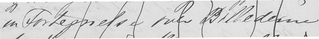en Fortegnelse over Billederne
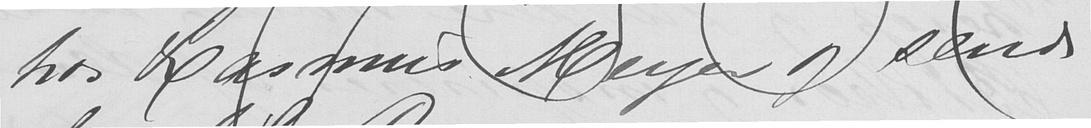hos Rasmus Meyer og sende
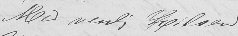Med venlig Hilsen
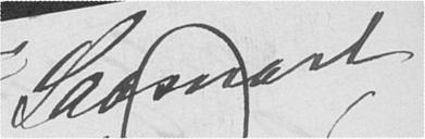Saasnart
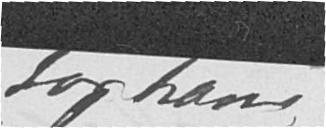for ham
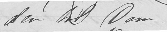den til Dem.
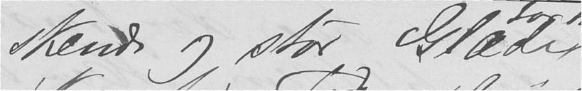skende og stor Glæde
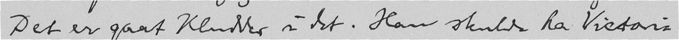Det er gaat Kludder i det. Han skulde ha Victoria
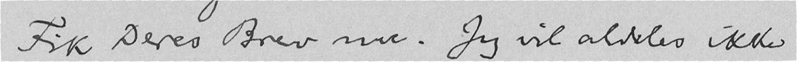Fik Deres Brev nu. Jeg vil aldeles ikke
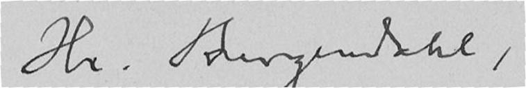Hr. Bergendahl,
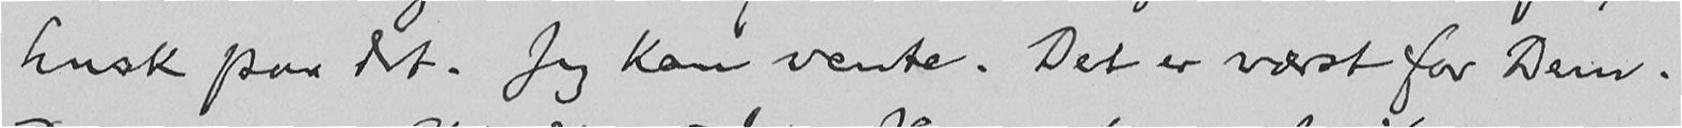husk paa det. Jeg kan vente. Det er værst for Dem.
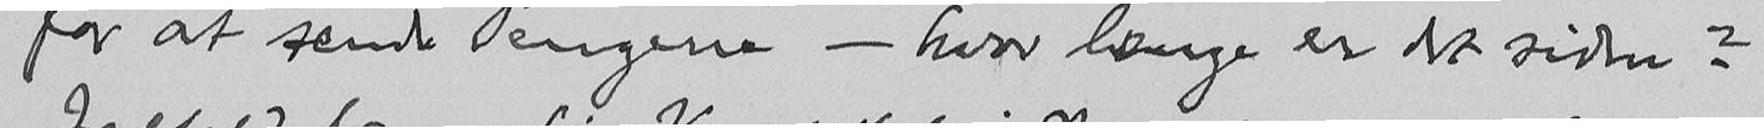for at sende Pengene - hvor længe er det siden?
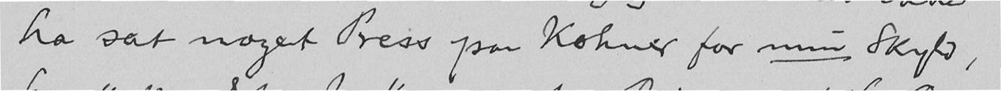ha sat noget Press paa Kohner for min Skyld,
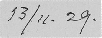13/11. 29.
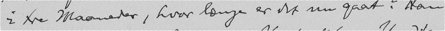i tre Maaneder, hvor længe er det nu gaat? Han
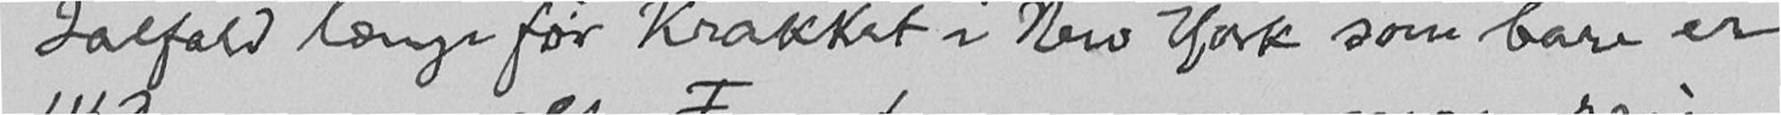Ialfald længe før Krakket i New York som bare er
